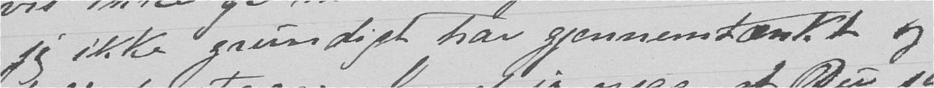jeg ikke grundigt har gjennemtænkt og
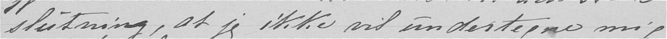slutning, at jeg ikke vil undertegne mig
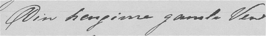Din hengivne gamle Ven
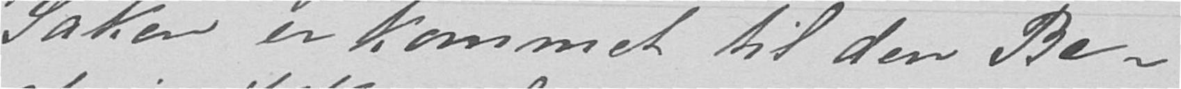Saken er kommet til den Be-
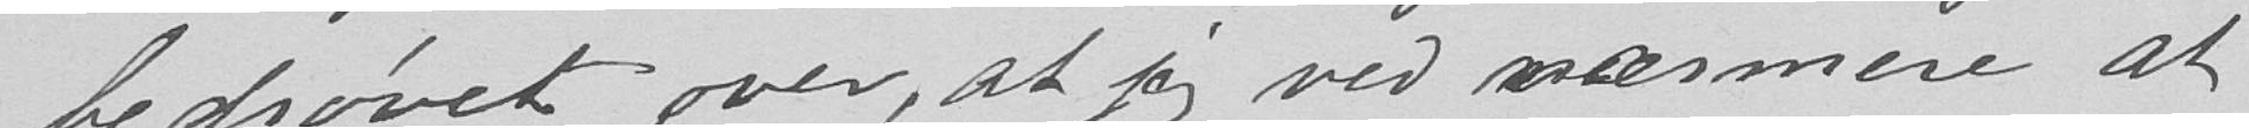bedrøvet over, at jeg ved nærmere at
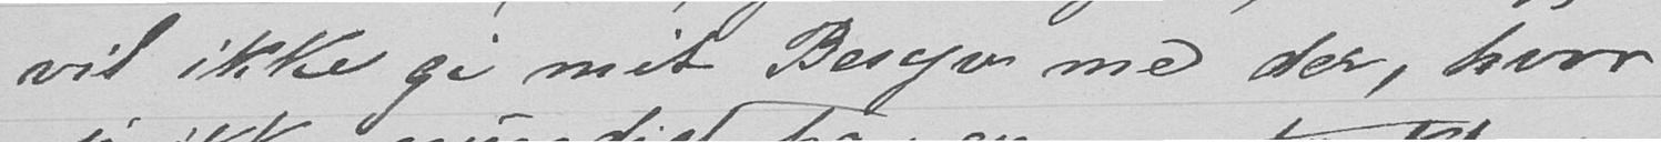vil ikke gi mit Besyv med der, hvor
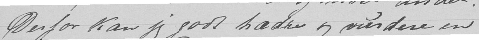Derfor kan jeg godt hædre og vurdere en
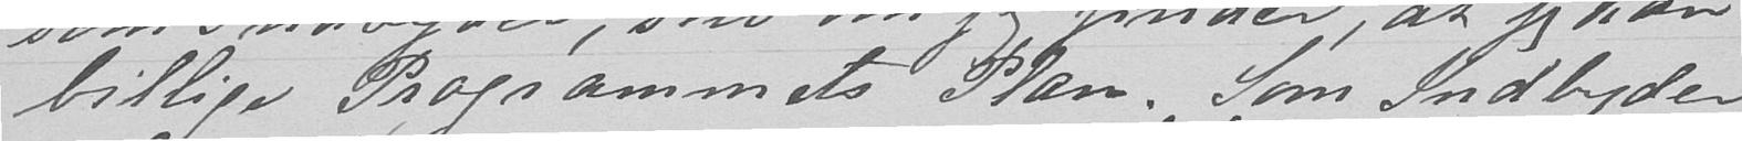billige Programmets Plan. Som Indbyder
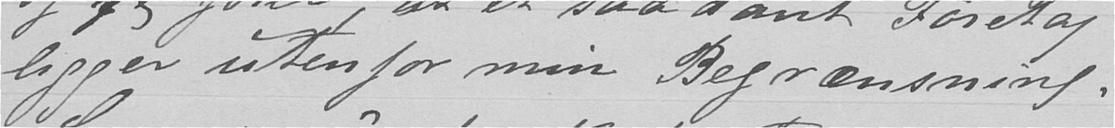ligger utenfor min Begrænsning.
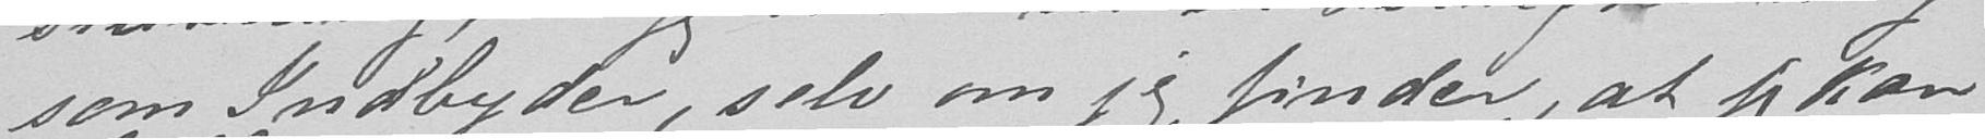som Indbyder, selv om jeg finder, at jeg kan
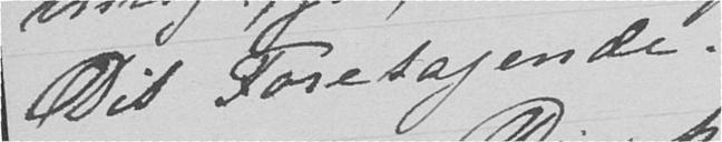Dit Foretagende.
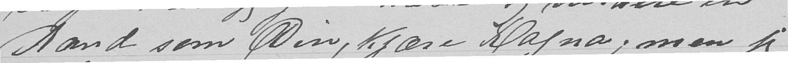Aand som Din, Kjære Ragna, men jeg
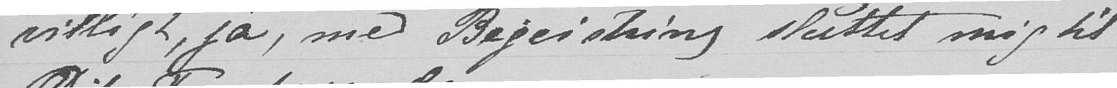villigt, ja, med Begeistring sluttet mig til
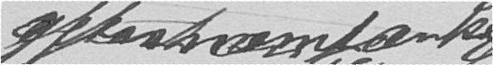gjennemtænke
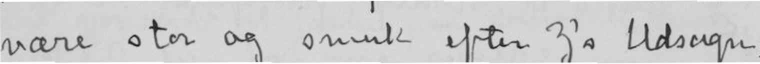være stor og smuk efter 3 Udsagn.
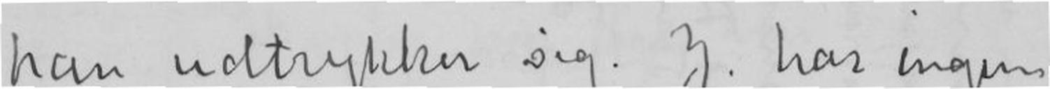han udtrykker sig. I har ingen
